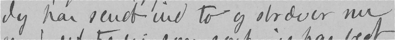Jeg har sendt ind to og stræver nu
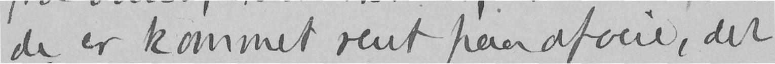de er kommet rent paa afveie, det
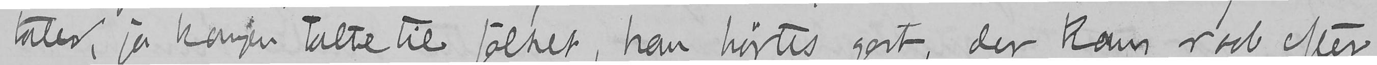taler, ja kongen talte til folket, han hørtes godt, der kom raab efter
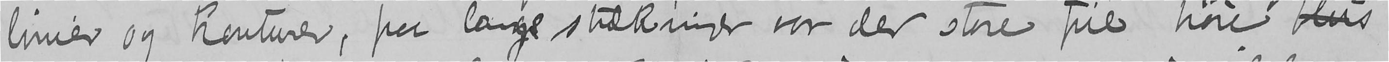linier og konturer, paa lange strækninger var der store fine høie blus
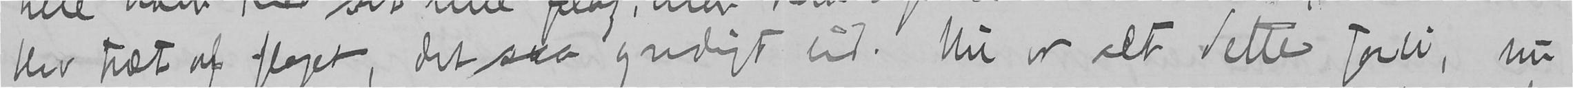blev træt af flaget, det saa grudigt ud. Nu er alt dette forbi, nu
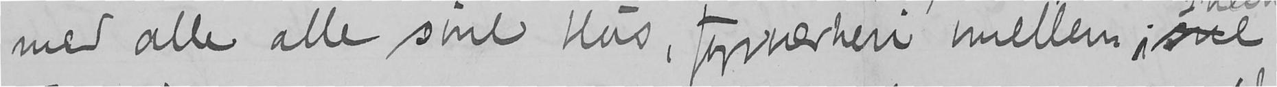med alle alle sine blus, fyrværkeri imellem; sne
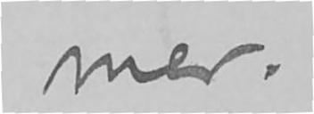mer.
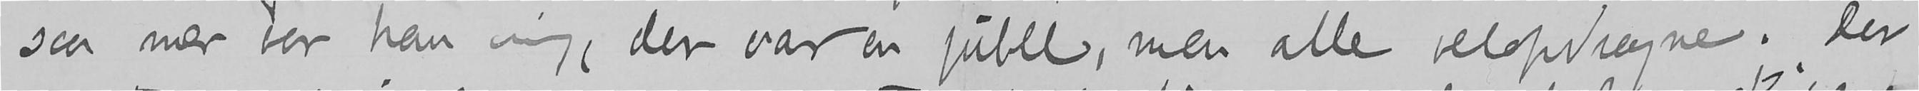saa nær var han mig, der var en jubel, men alle velopdragne. Der
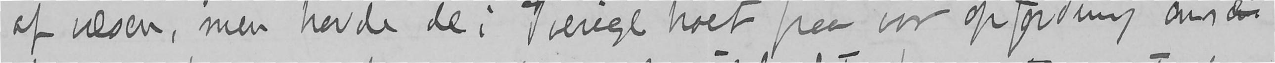af væsen, men havde de i Sverige troet paa vor opfordring om den
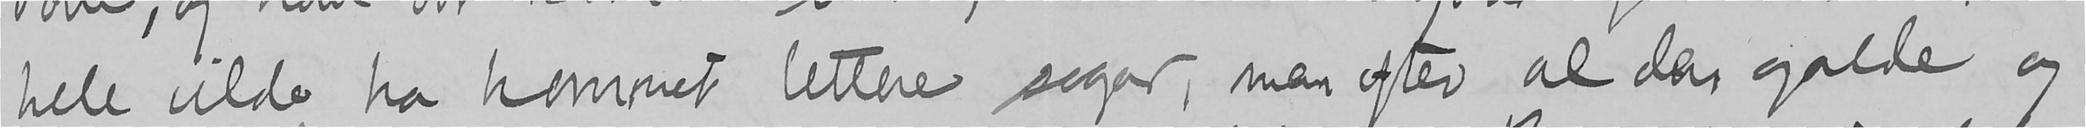hele vilde ha kommet lettere sogar, men efter al den galde og
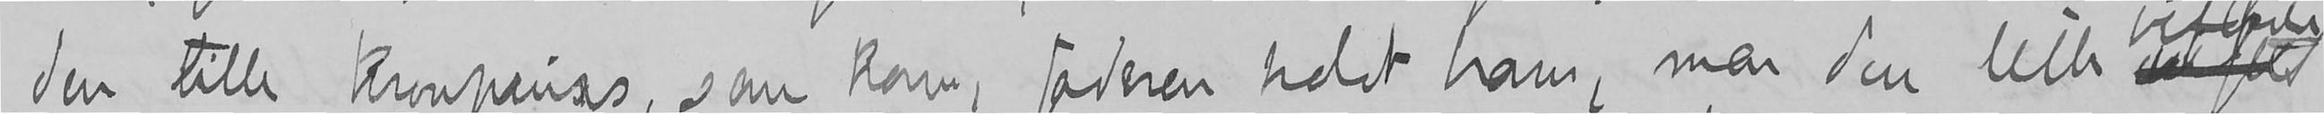den lille Kronprins, som kom, faderen holdt ham, men den lille viftet
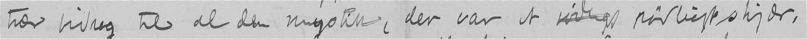trær bidrog til al den mystik, der var et rødeligt rødligt skjær,
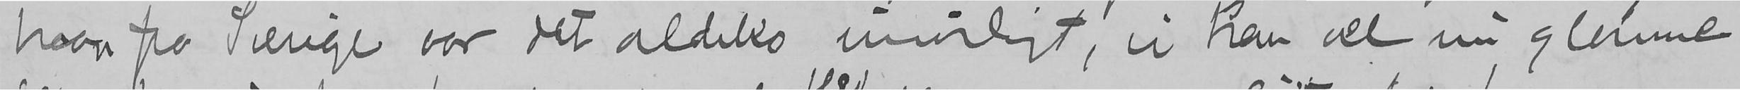haan fra Sverige var det aldeles umuligt, vi kan vel nu glemme
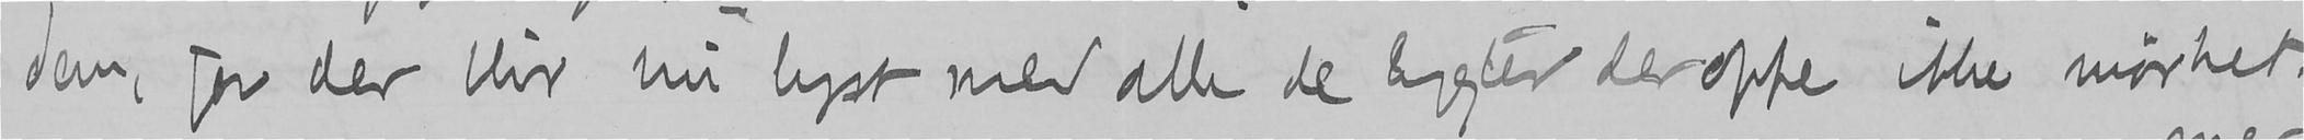dem, for der blir nu lyst med alle de lygter deroppe ikke mørket.
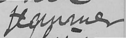flammer
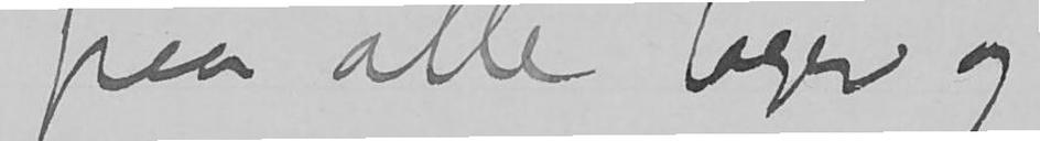paa alle tager og
